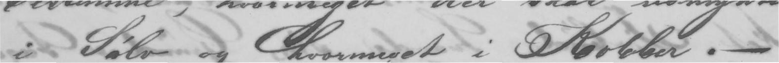i Sølv og hvormeget i Kobber. -
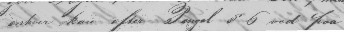enhver efter Pengel §6 ved paa
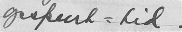opspurt-tid.
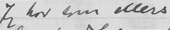Jeg har som ellers
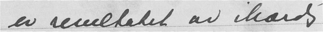er resultatet av ihærdig
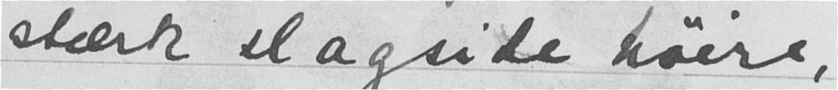stærk slagside høire,
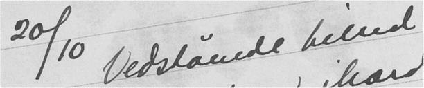20/10 Vedstående billed
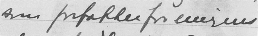som forfatterforeningens
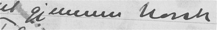ut gjennom Norsk
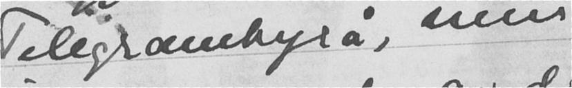Telegrambyrå, men
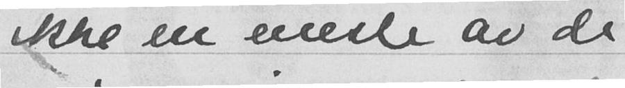ikke en eneste av de
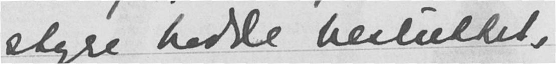styre hadde besluttet,
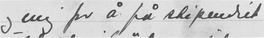og mig for å få stipendiet
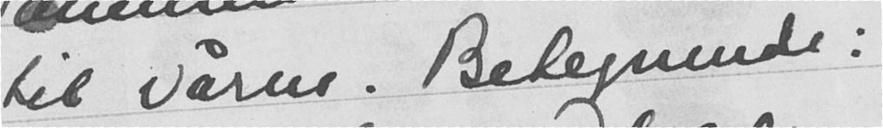til våren. Betegnende:
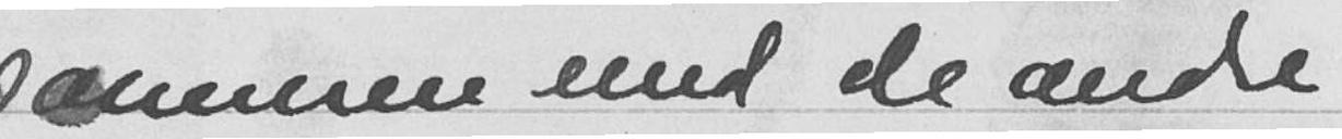sammen med de andre
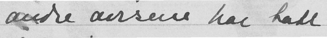andre avisene har tatt
